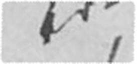ere
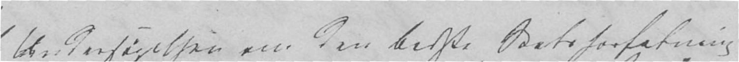Undersøgelsen om den bedste Statsforfatning
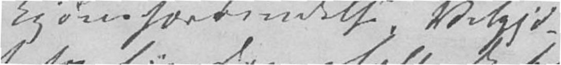Kjønsforbindelse, Velgjø-
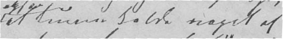at kunne kalde noget af
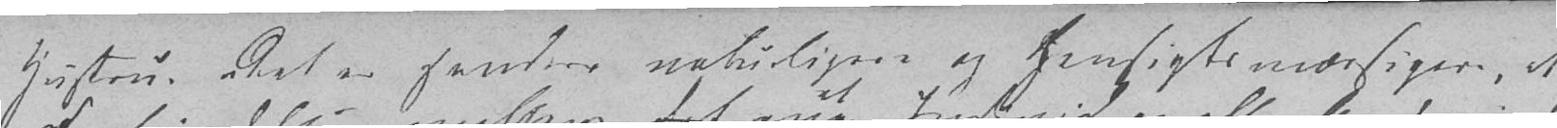Hustru. Det er sandtes naturligere og hensigtsmæssigere, at
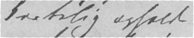kortelig opholde
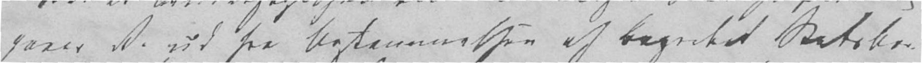gaaer A. ud fra Bestemmelsen af Begrebet Statsbor-
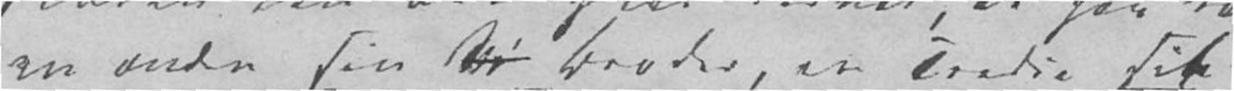en anden sin Sø Broder, en Tredie sit
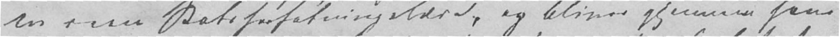en reen Statsforfatningslære, og bliver gjennem hans
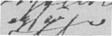ogsaa
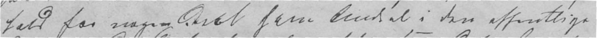fald for nogen Deel have Andeel i den offentlige
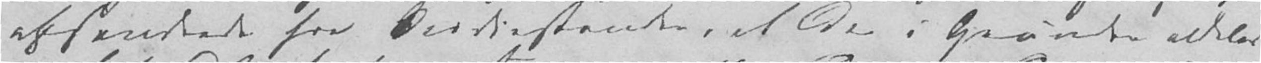afsondrede fra Trediestanden, at der i Grunden aldeles
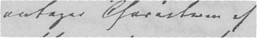antager Characteer af
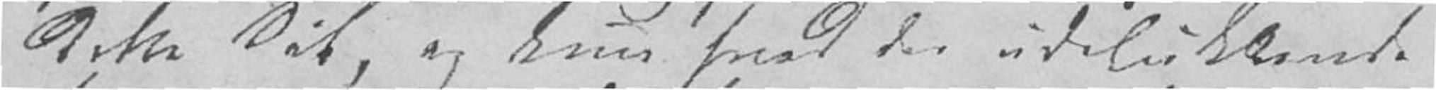dette Sit, og kun hvad der udelukkende
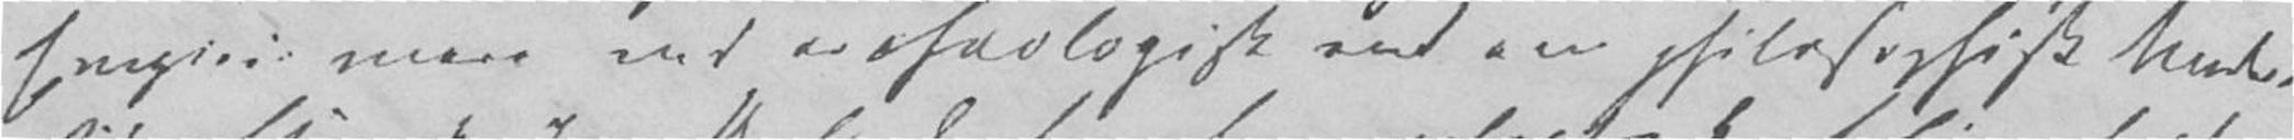Empirie mere en archeologisk end en philosophisk Under-
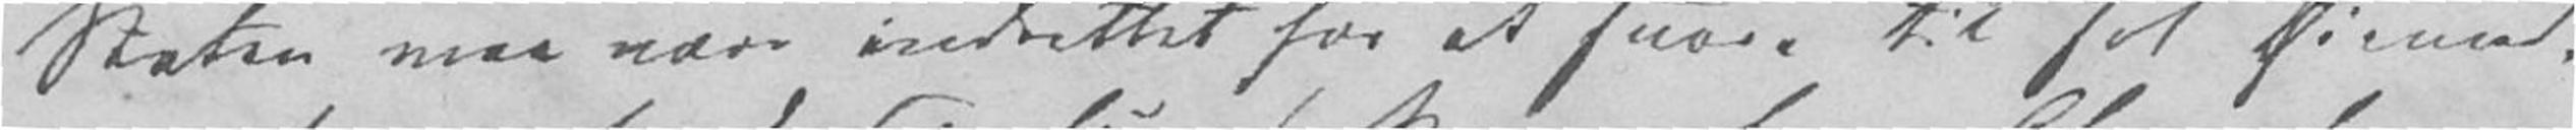Staten maa være indrettet for at svare til sit Øiemed,
